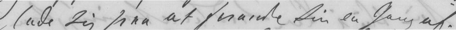lade sig paa at forandre sin en Gang af-
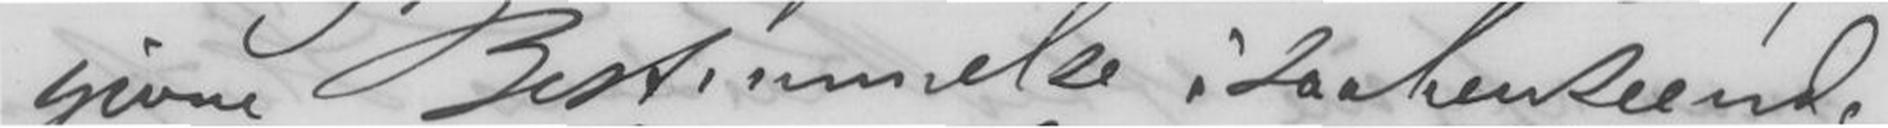givne Bestemmelse isaahenseende.
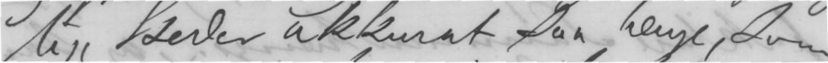lige Steder akkurat saa længe, som
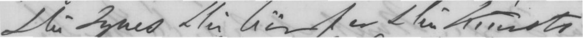Du synes Du bør for Din Kunsts
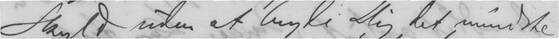Skyld uden at bryde Dig det mindste
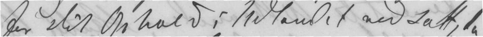for Dit Ophold i Udlandet nedsatt, da
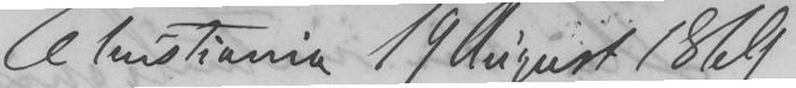Christiania 19 August 1869.
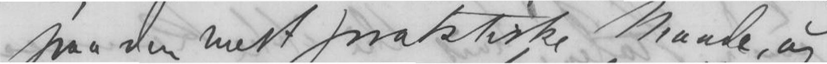paa den mest praktiske Maade, og
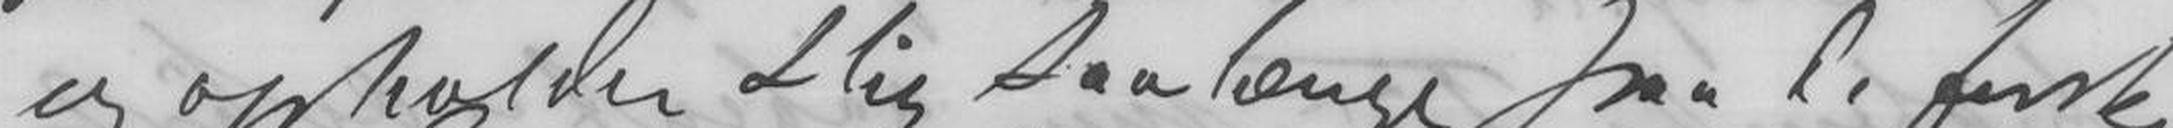og opholder Dig saalænge paa de forskjel-
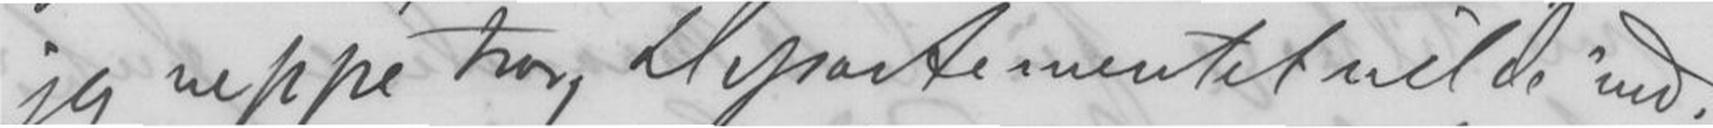jeg neppe tror, Departementet vilde ind-
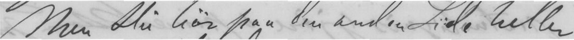Men Du bør paa den anden Side heller
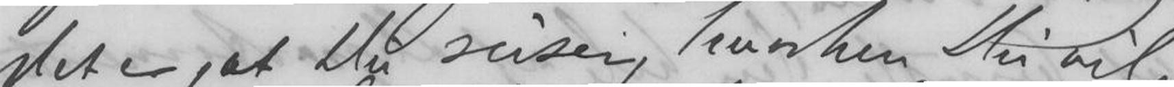det er, at Du reiser hvorhen Du vil
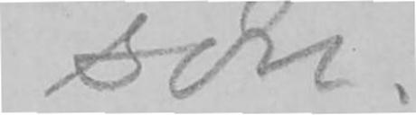son.
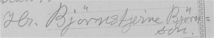Hr. Bjørnstjerne Bjørn-
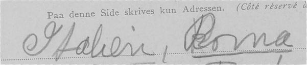Italien, Roma
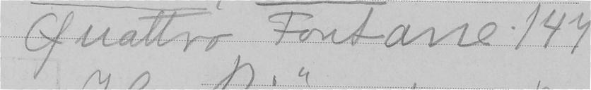Quattero Fontane 144
